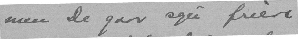men De gaar sgu friere
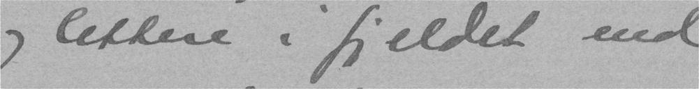og lettere i fjeldet end
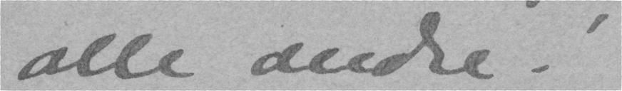alle andre!
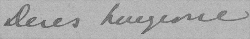Deres hengivne
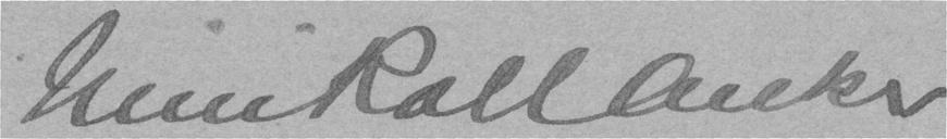Nini Roll Anker
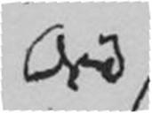Ord
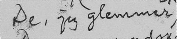De, jeg glemmer
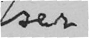ser
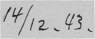14/12. 43.
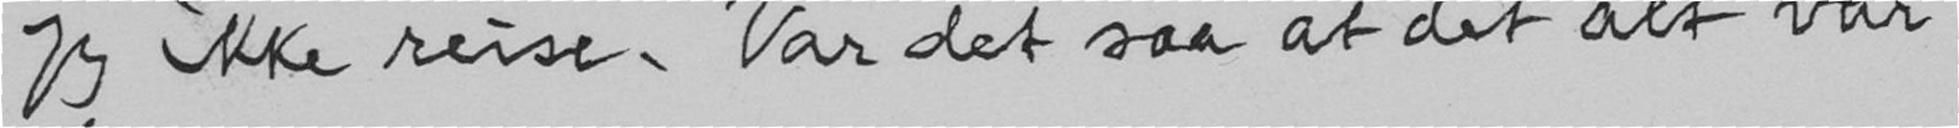jeg ikke reise. Var det saa at det alt var
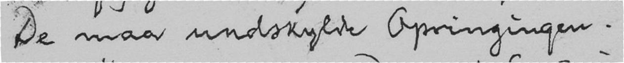De maa undskylde Opringingen.
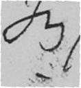Jeg
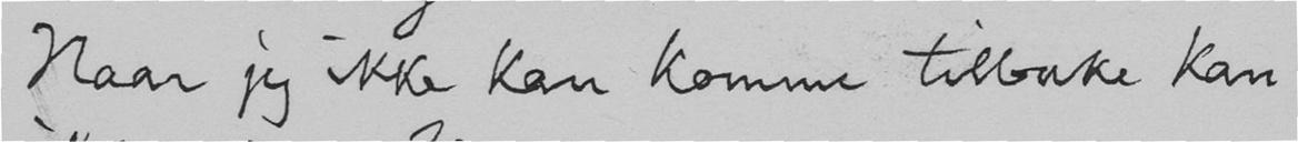Naar jeg ikke kan komme tilbake kan
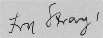Fru Stray,
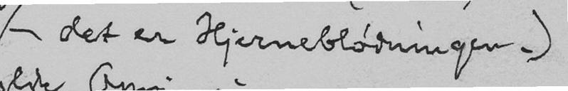- det er Hjerneblødningen.)
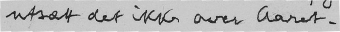utsætt det ikke over Aaret.
